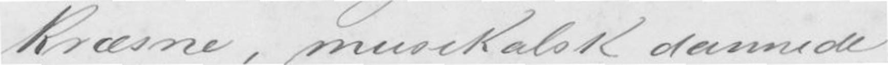kræsne, musikalsk dannede,
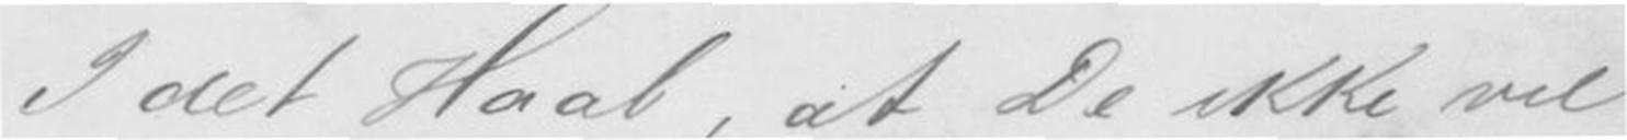I det Haab, at De ikke vil
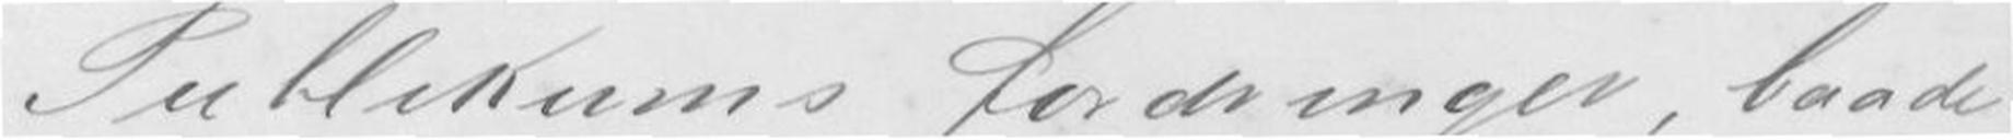Publikums fordringer, baade
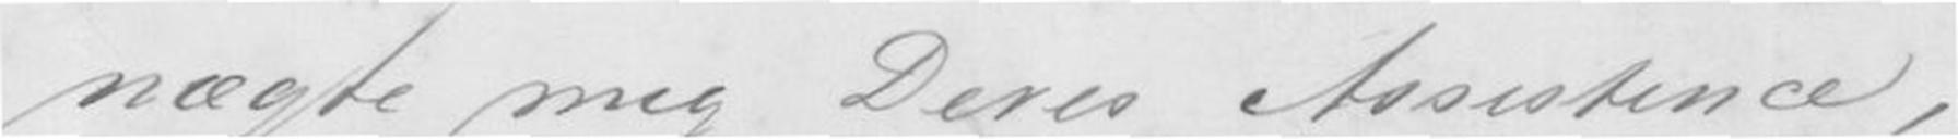nægte mig Deres Assistance,
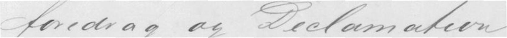foredrag og Declamation
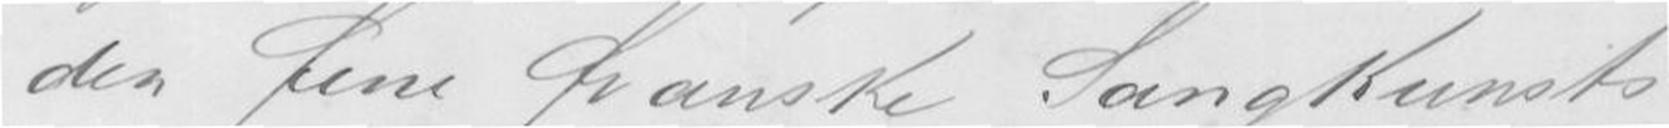den fine franske Sangkunsts
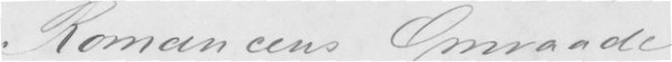Romancens Omraade.
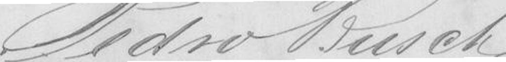Pedro Busch
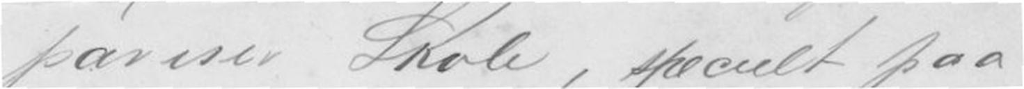pariser Skole, specielt paa
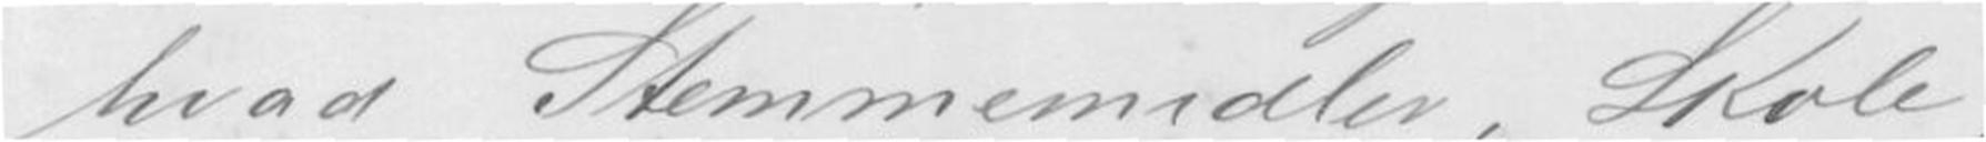hvad Stemmemidler, Skole,
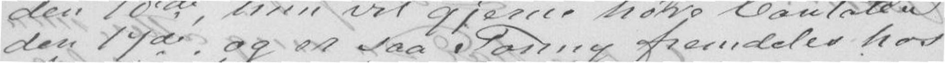den 17de, og er saa Tonny fremdeles hos
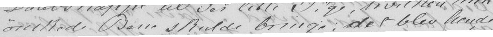ønskede Bene skulde bringe; det blev hende
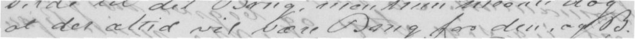at der altid vil være Brug for den, og B.
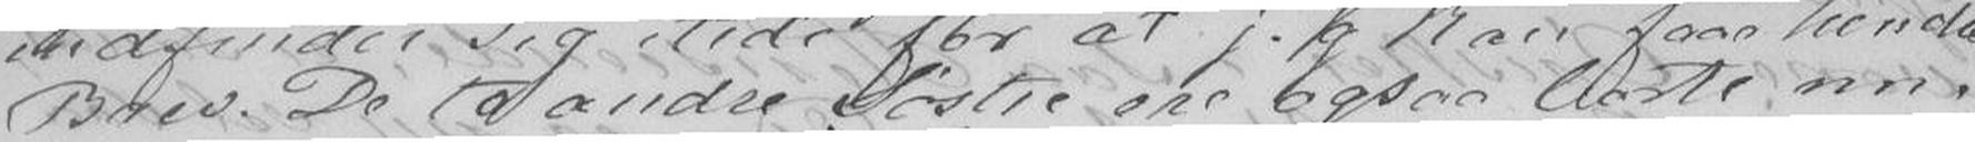Brev. De to andre Søstre ere ogsaa borte nu,
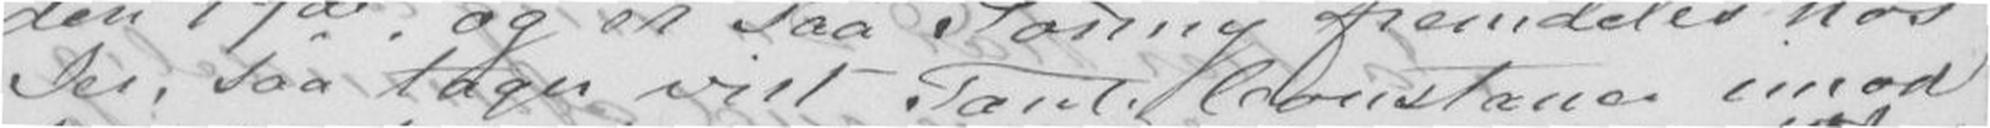Jer, saa tager vist Tante Constance imod
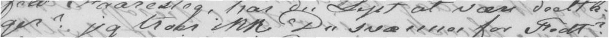ger? jeg troer ikke Du sværmer for Fedt?
